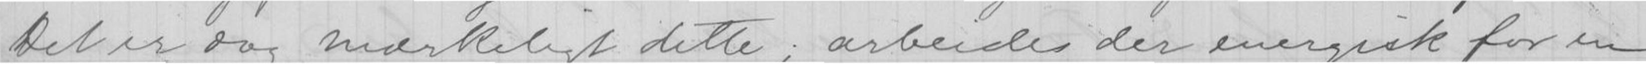Det er dog mærkelig dette; arbeides der energisk for en
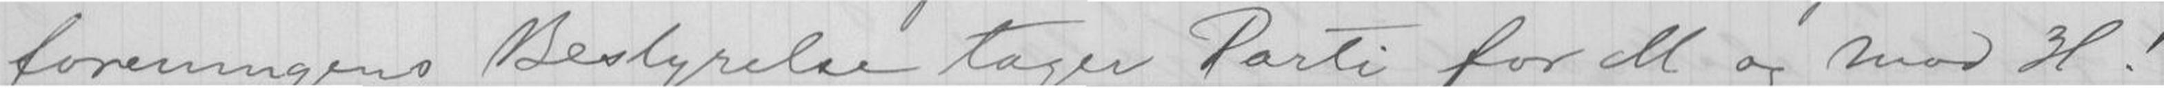foreningens Bestyrelse tager Parti for M og mod H!
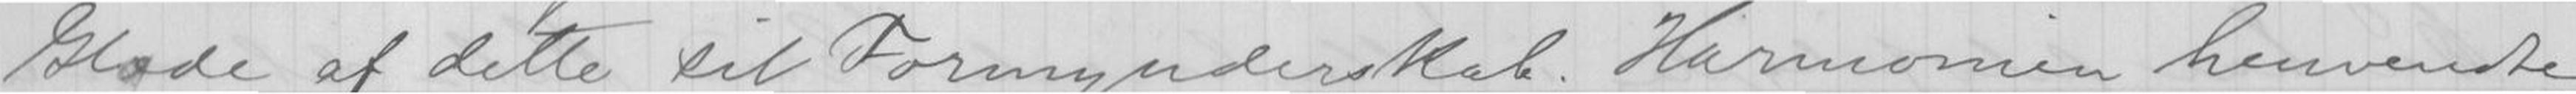Glæde af dette sit Formynderskab. Harmonien henvendte
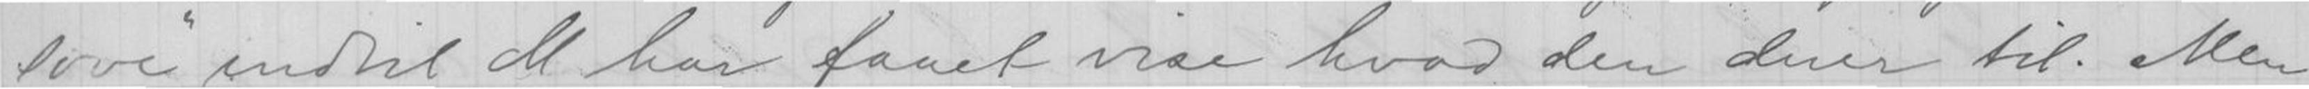sove indtil M har faaet vise hvad den duer til. Men
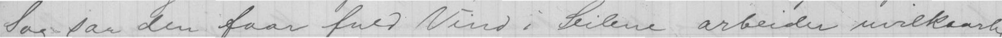Sag saa den faar fuld Vind i Seilene, arbeider uvilkaarlig
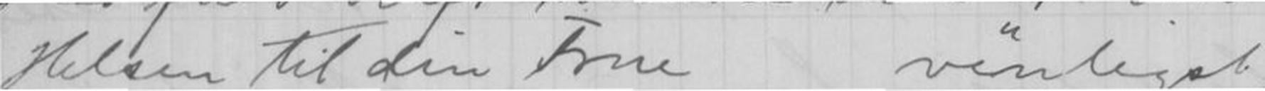Hilsen til din Fruevenligst
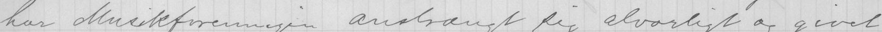har Musikforeningen anstrængt sig alvorligt og givet
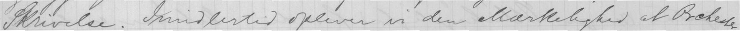Skrivelse. Imidlertid oplever vi den Mærkelighed at Orchester-
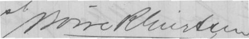Børre R Giertsen
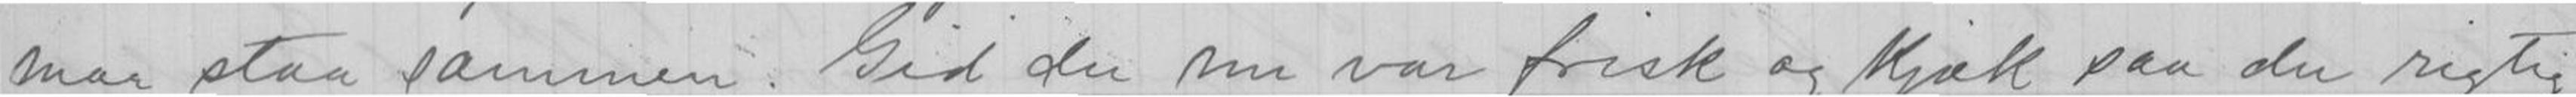maa staa sammen. Gid du nu var frisk og kjæk saa du rigtig
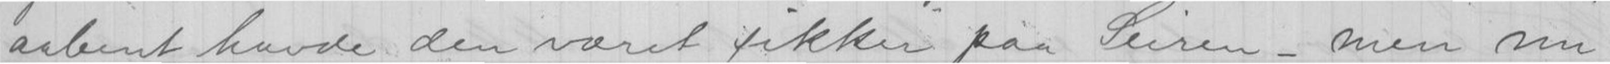aabent, havde den været sikker paa Seiren - men nu
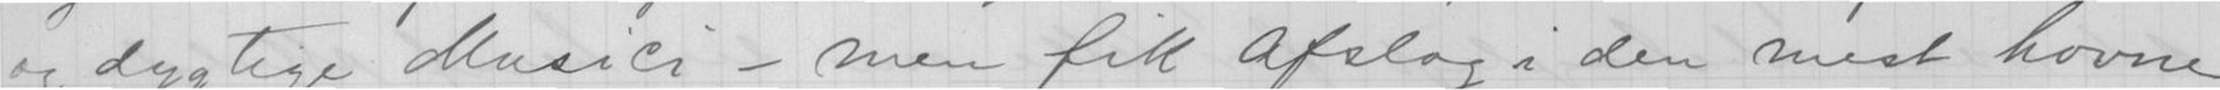og dygtige Musici - men fik Afslag i den mest hovne
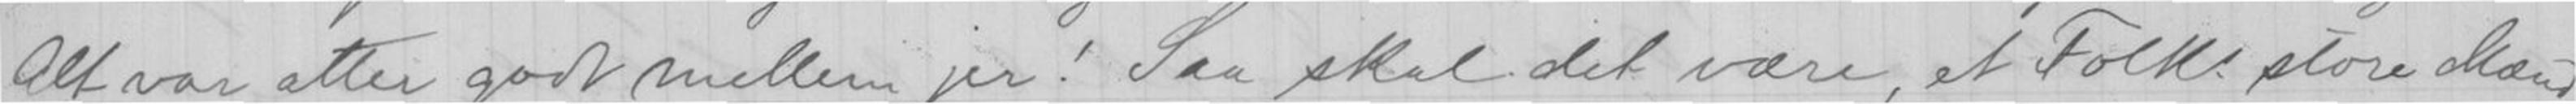Alt var atter godt mellem jer! Saa skal det være, et Folks store Mænd
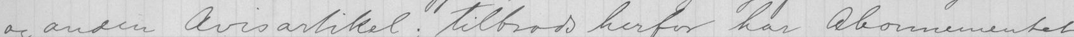og anden Avisartikel; tiltrods herfor har Abonnementet
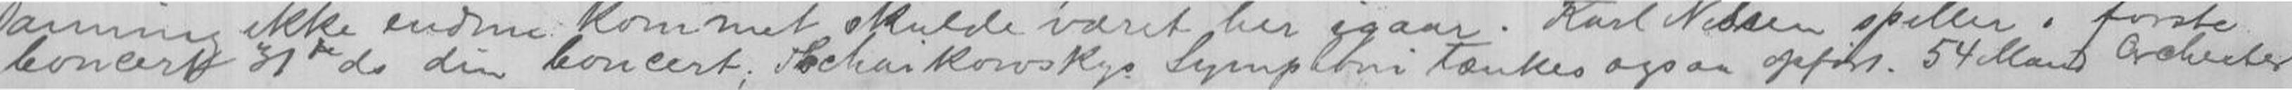Concert 31de ds din Concert; Tschaikowskys Symphoni tænkes ogsaa opført. 54 Mand Orchester.
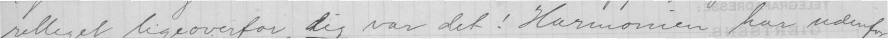rettiget ligeoverfor dig var det! Harmonien har udenfor
